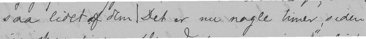saa lidet af dem | Det er nu nogle timer, siden
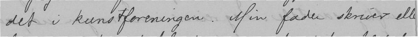det i kunstforeningen. Min fader skriver eller
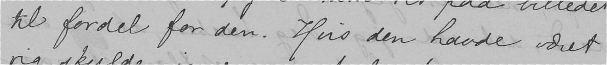til fordel for den. Hvis den havde været
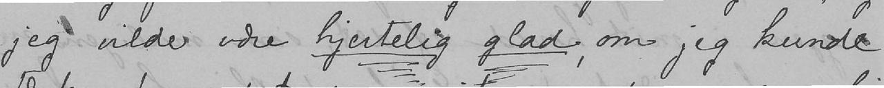jeg vilde være hjertelig glad, om jeg kunde
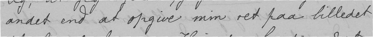andet end at opgive min ret paa billedet
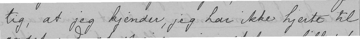tig, at jeg kjender, jeg har ikke hjerte til
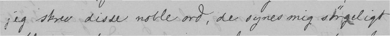jeg skrev disse noble ord, de synes mig sørgeligt
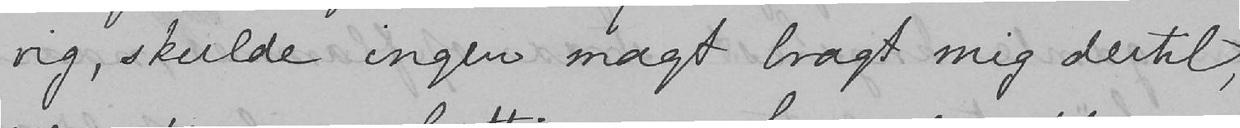rig, skulde ingen magt bragt mig dertil,
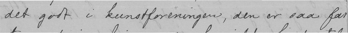det godt i kunstforeningen, den er saa fat-
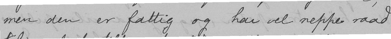men den er fattig og har vel neppe raad
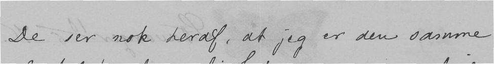De ser nok heraf, at jeg er den samme
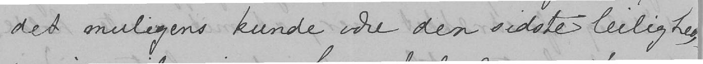det muligens kunde være den sidste leilighed,
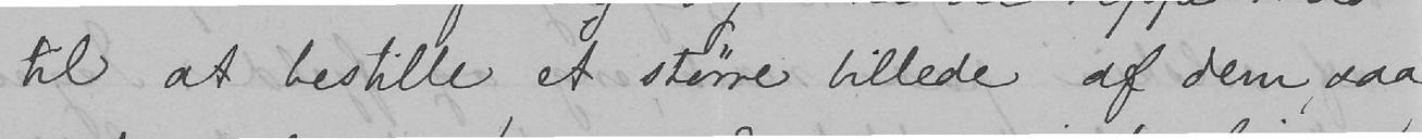til at bestille et større billede af dem, saa
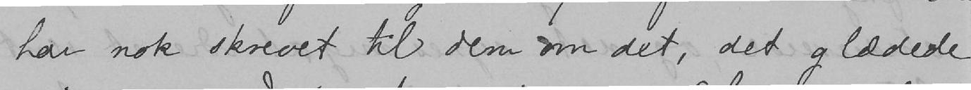har nok skrevet til dem om det, det glædede
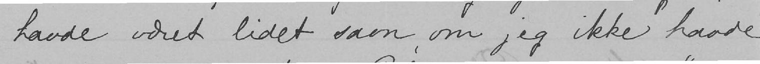havde været lidet savn, om jeg ikke havde
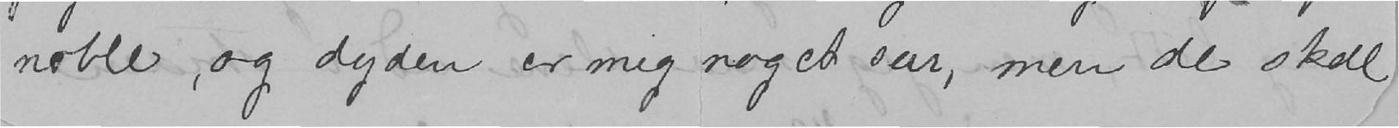noble, og dyden er mig noget sær, men de skal
